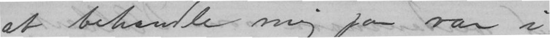at behandle mig paa var i
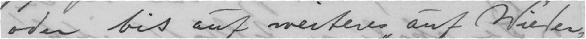oder bis auf weiteres "auf Wieder-
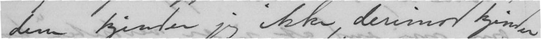dem kjender jeg ikke, derimod kjender
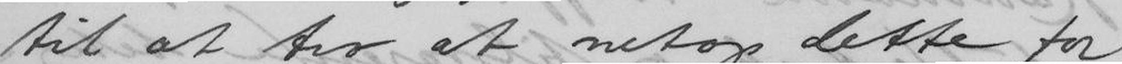til at tro at netop dette for
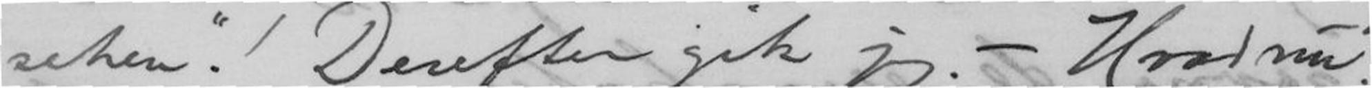sehen"! Derefter gik jeg. - Hvad nu?
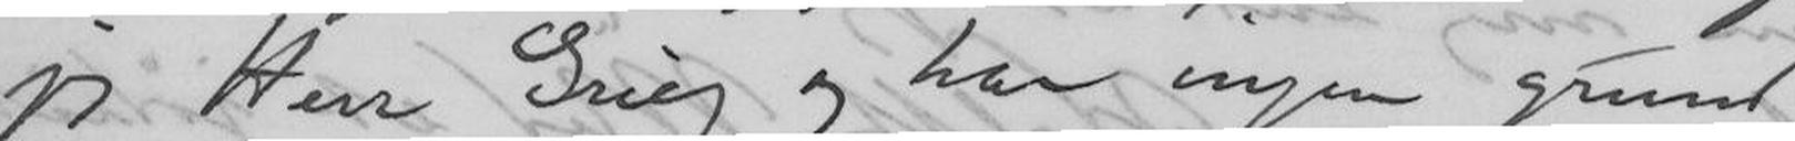jeg Herr Grieg og har ingen grund
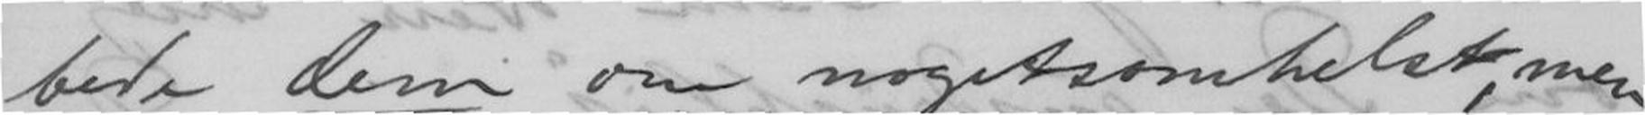bede dem om nogetsomhelst, men
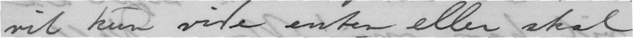vil kun vide enten eller, skal
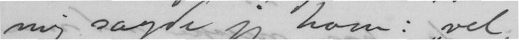mig sagde jeg ham: vel,
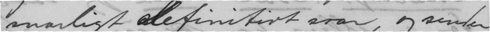snarligt definitivt svar, og sender
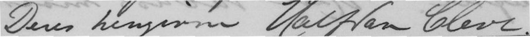Deres hengivne Halfdan Cleve.
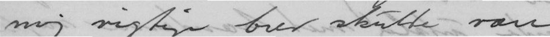mig vigtige brev skulde være
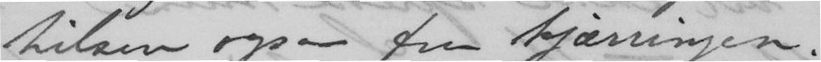hilsen ogsaa fra kjærringen.
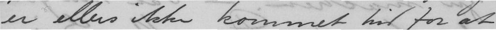er ellers ikke kommet hid for at
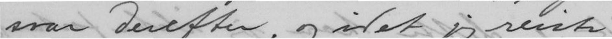svar derefter, og idet jeg reiste
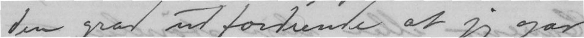den grad udfordrende at jeg gav
